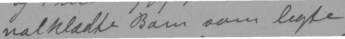nalklædte Barn som legte
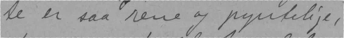te er saa rene og pyntelige,
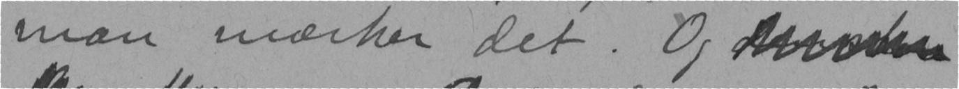man mærker det. Og
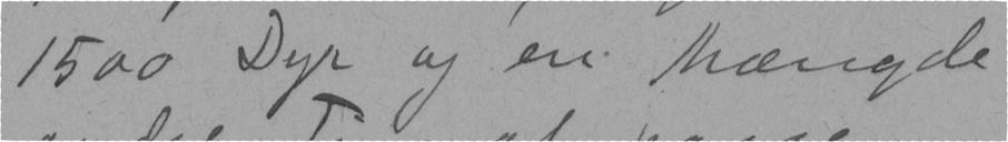1500 Dyr og en Mængde
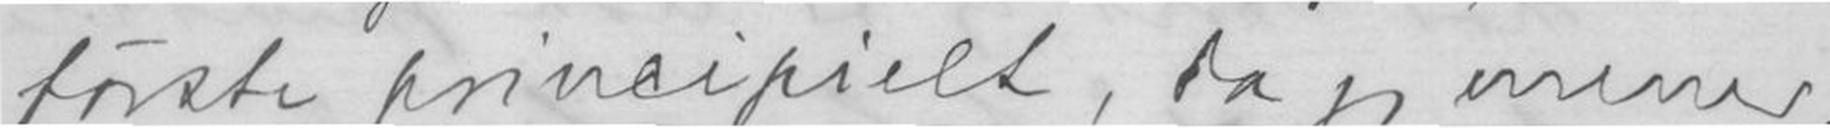første principielt, da jeg mener,
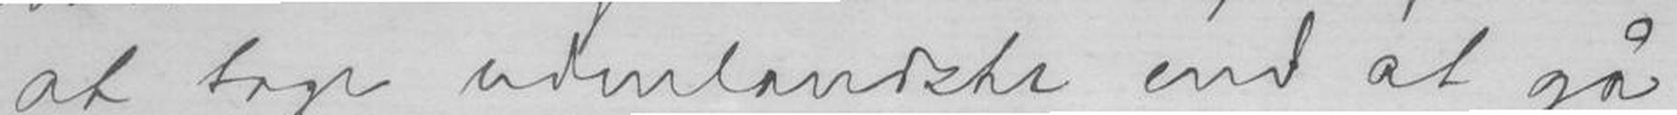at tage udenlandske end at gå
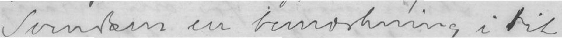Svendsen en bemærkning i dit
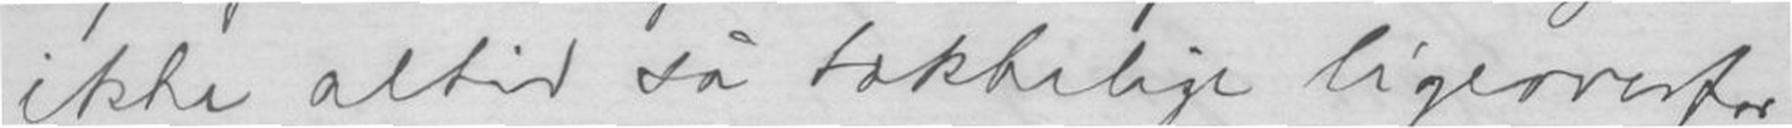ikke altid så tækkelige ligeovenfor
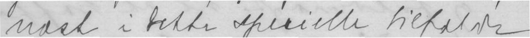næst i dette specielle tilfældet,
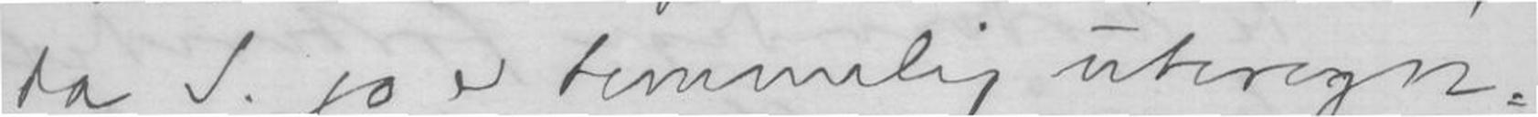da S. jo er temmelig uberegn-
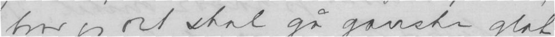tror jeg det skal gå ganske glat.
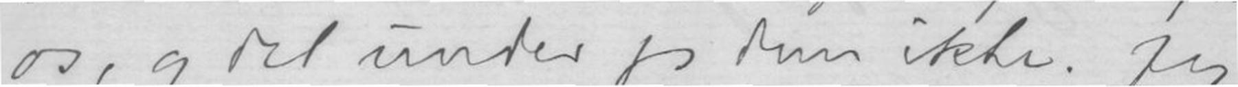os, og det under jeg dem ikke. Jeg
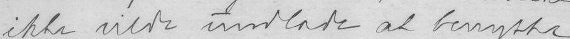ikke vilde undlade at benytte
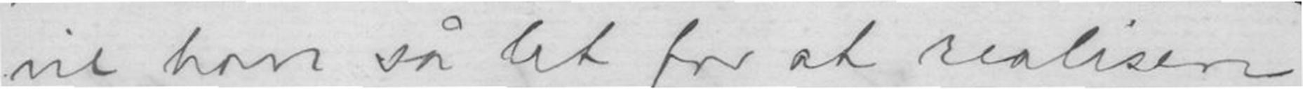vil have så let for at realisere
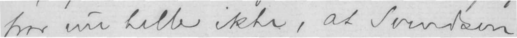tror nu heller ikke, at Svendsen
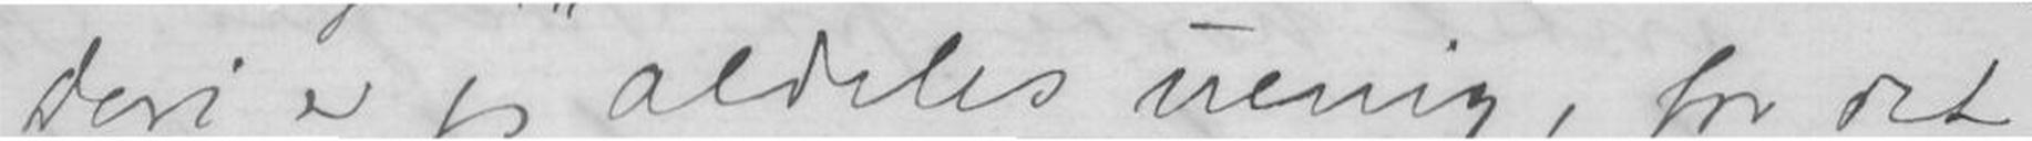deri er jeg aldeles uenig, for det
